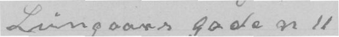Lundgaars Gade nr 11
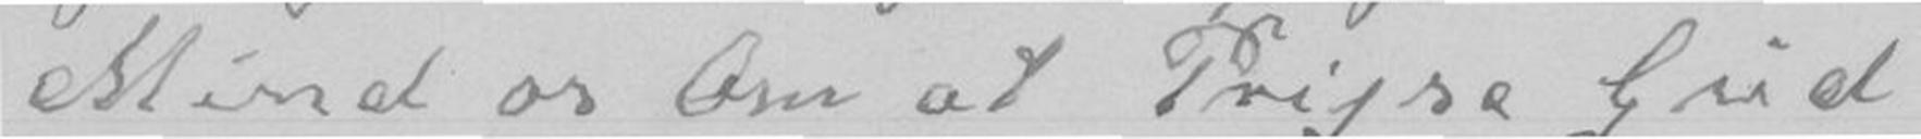Mind os Om at Prisse Gud
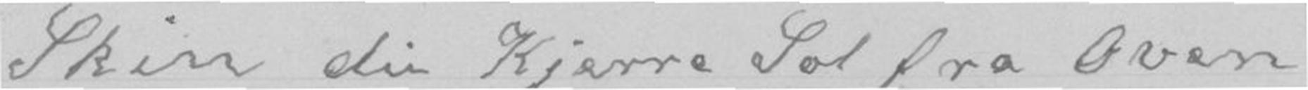Skin du Kjerre Sol fra Oven
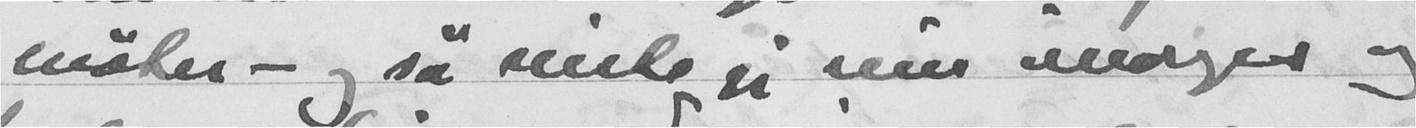møter - og så viste jeg min ieriges og
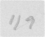119
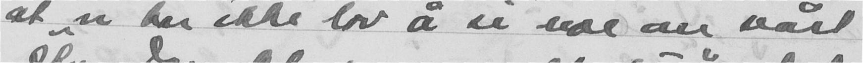at "vi har ikke lov å si noe om vårt
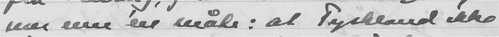mer enn en måte; at Tyskland ikke
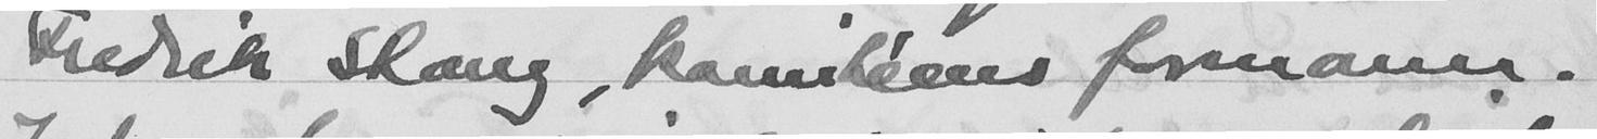Fredrik Stang, komitéens formann.
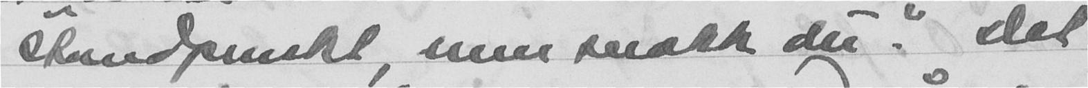standpunkt, men snakk du. Det
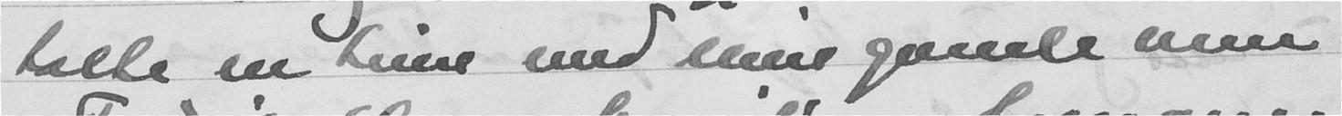talte en time med min gamle venn
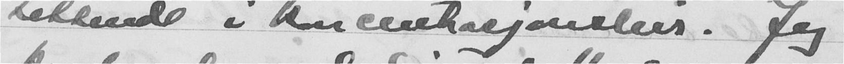sittende i koncentrasjonsleir. Jeg
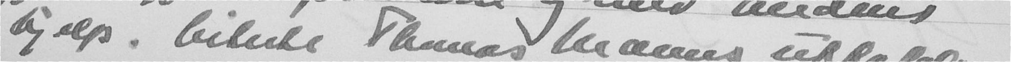hjælp. Siterte Thomas Manns uttalelse
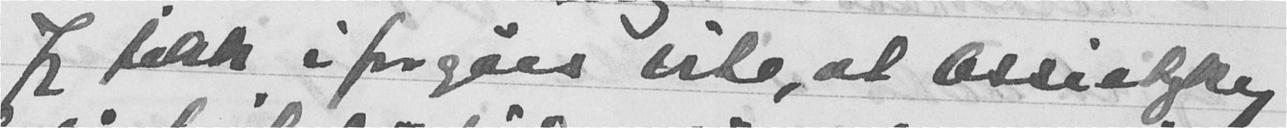Jeg fikk i forgårs vite, at Ossietzky
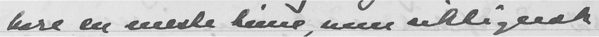bare en eneste time, men riktignok
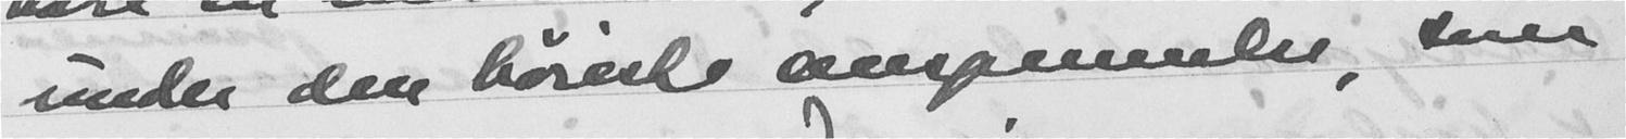under den høiese anspennelse, som
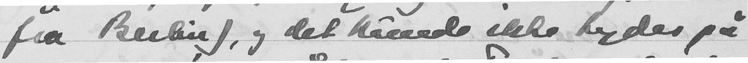fra Berlin), og det kunde ikke tydes på
